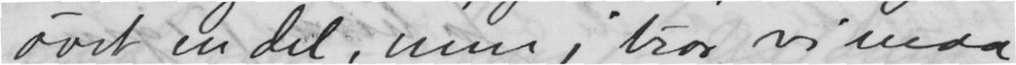øvet en del, men j tror vi maa
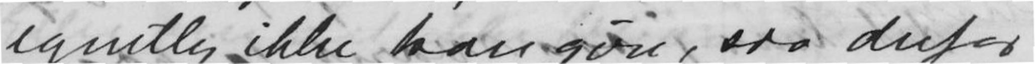egentlig ikke kan gøre, saa derfor
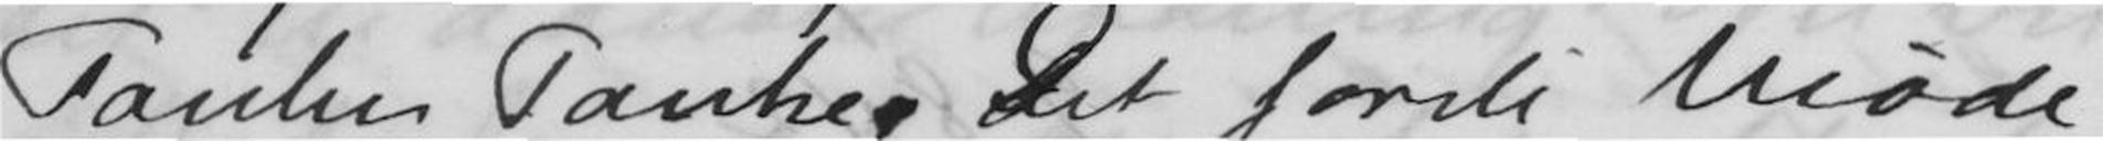Tankes Tanke. Det første Møde
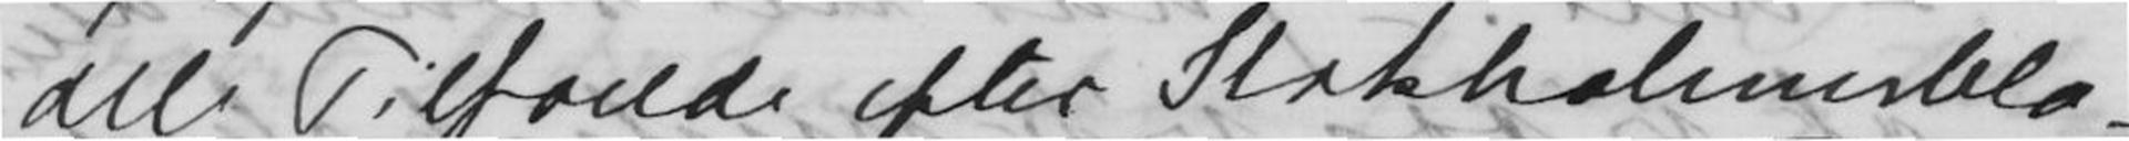alle Tilfaelde efter Stockholmsbla-
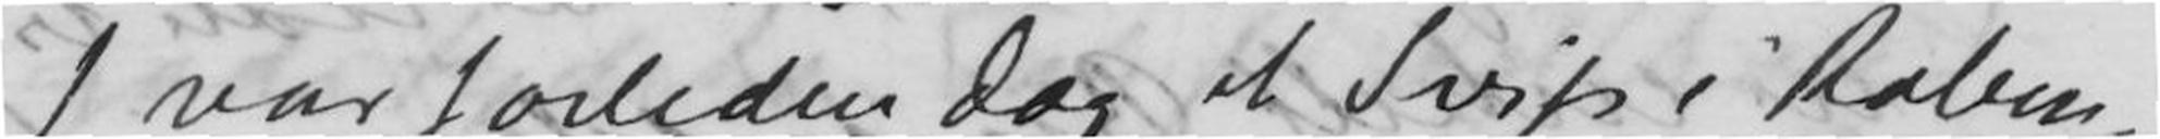J var forleden Dag et Svip i Køben-
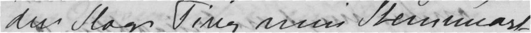den Slags Ting mine Stemmeast
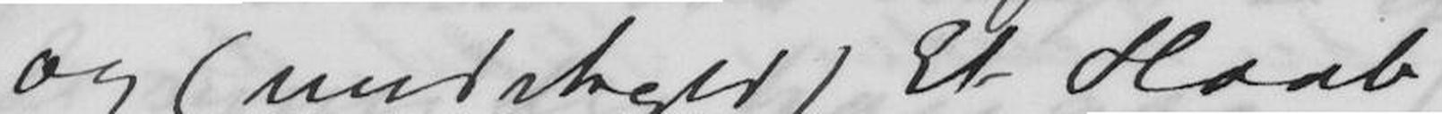og (undskyld) Et Haab! -
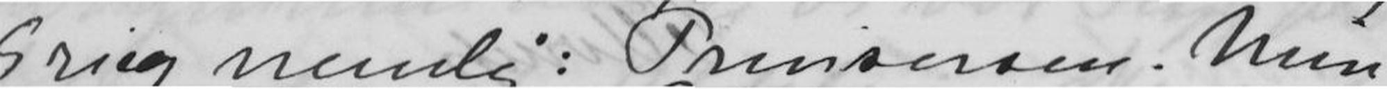Grieg nemlig: Prinsessen. Min
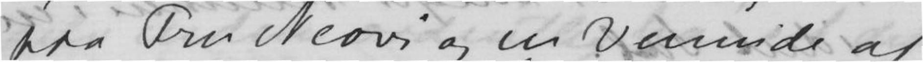paa Fru Neovi og en Veninde af
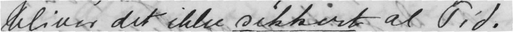bliver det ikke sikkert al Tid.-
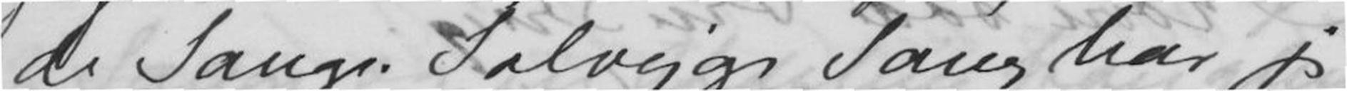de Sange. Solvejgs Sang har jeg
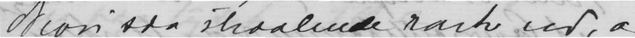Neovi saa straalende rask ud, og
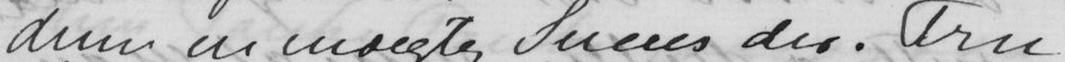derne en maegtig Succes der. Fru
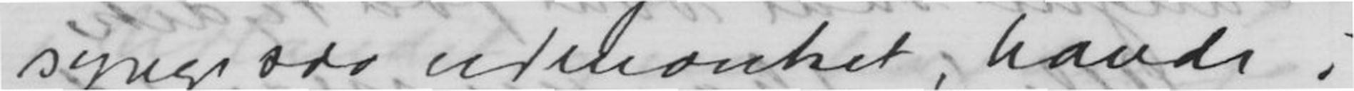synge saa udmaerket, havde i
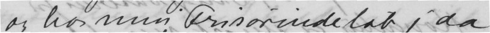og hos min Frisørinde løb j da
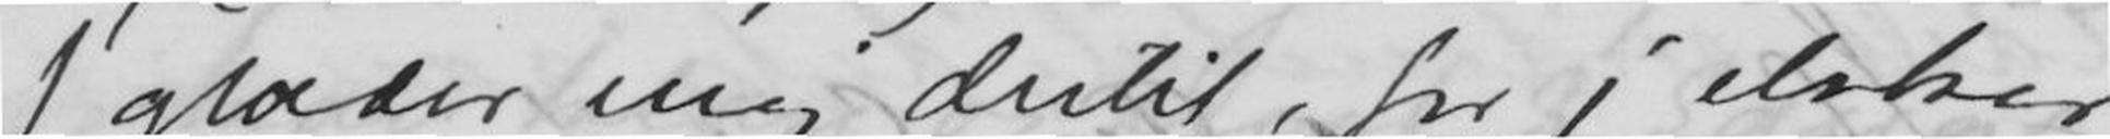J glaeder mig dertil, for j elsker
